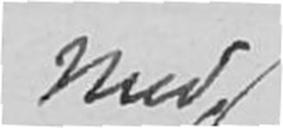med
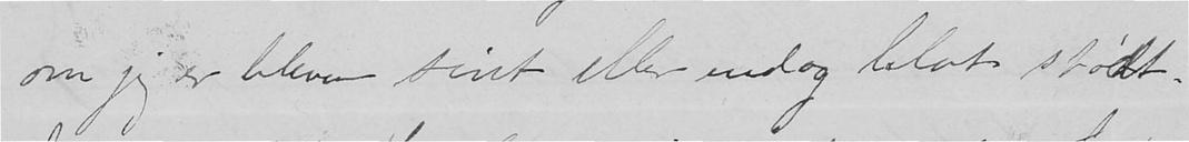om jeg er bleven sint eller endog blot stødt.
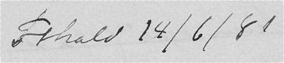Frhald 14/6/81
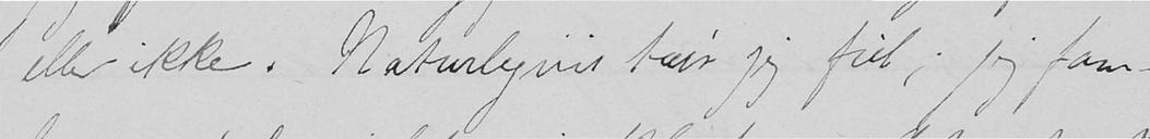eller ikke. Naturligvis tae'r jeg feil; jeg fam-
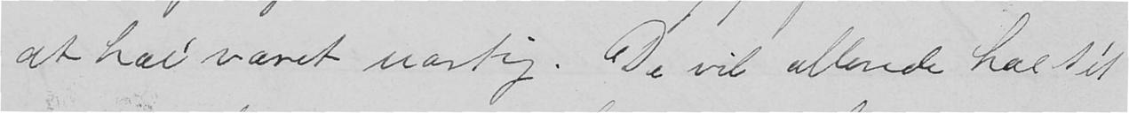at hae' været uartig. De vil allerede hae sét
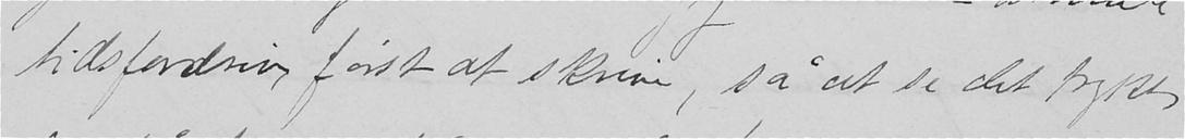tidsfordriv, først at skrive, så at se det trykt,
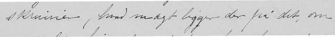skriverier, hvad magt ligger der på det, om
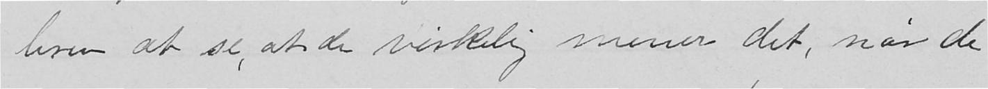brev at se, at de virkelig mener det, når de
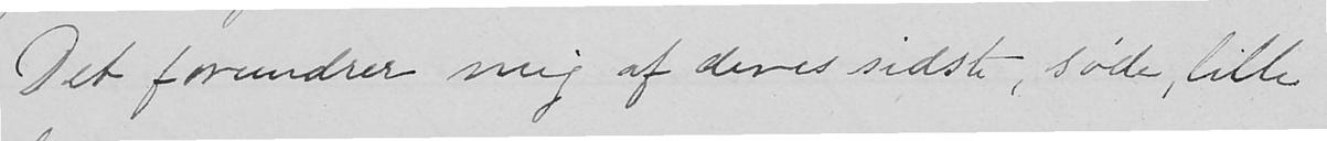Det forundrer mig af deres sidste, søde, lille
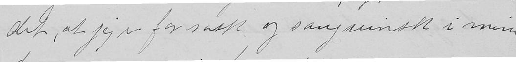det, at jeg er for rask og sangvinsk i mine
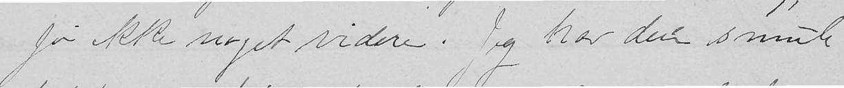jo ikke noget videre. Jeg har den smule
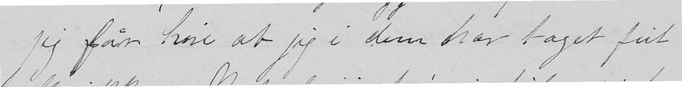jeg får høre at jeg i dem har taget feil
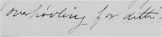overhøvling for dette
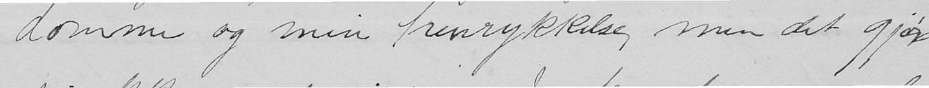domme og min henrykkelse, men det gjør
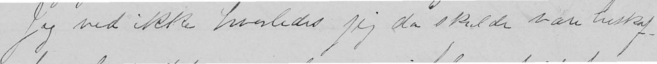Jeg ved ikke hvorledes jeg da skulde være beskaf-
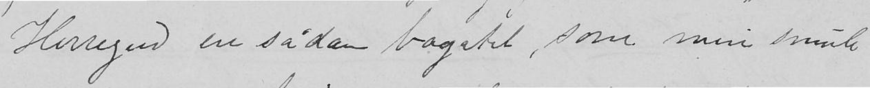Herregud en sådan bagatel, som min smule
